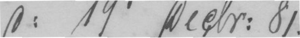C: 19 Decbr. 81.
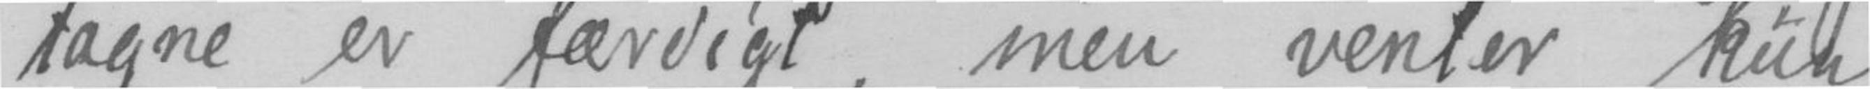tagne" er færdige, men venter kun
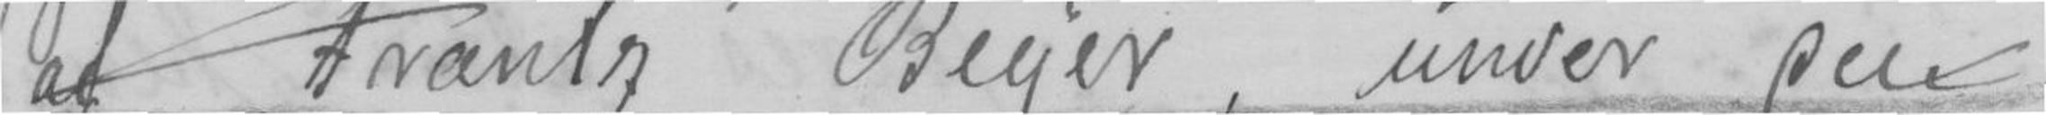af Frantq Beyer, under den
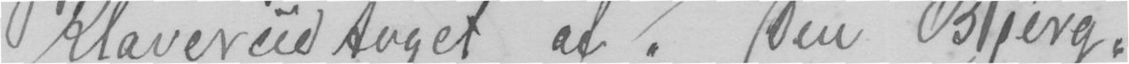Klaverudtoget af "Den Bjerg-
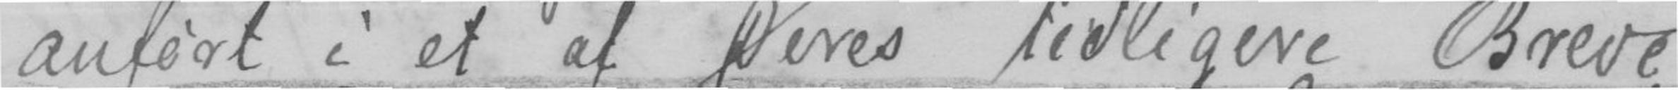anført i et af Deres tidligere Breve
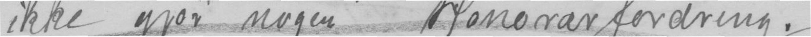ikke gjør nogen Honorar fordring.
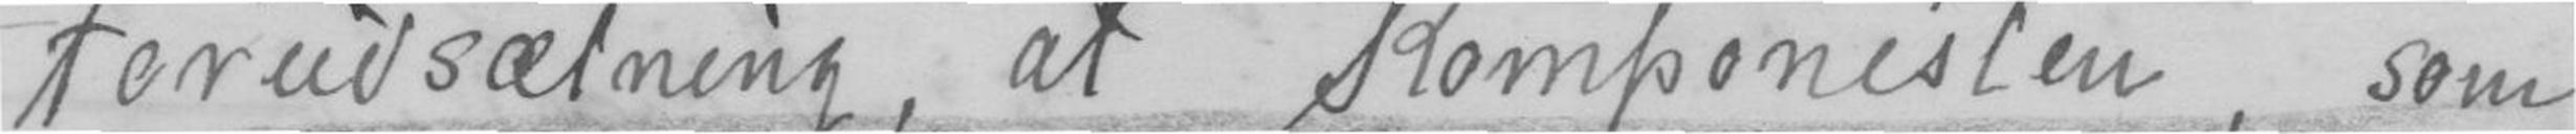Forudsætning, at Komponisten, som
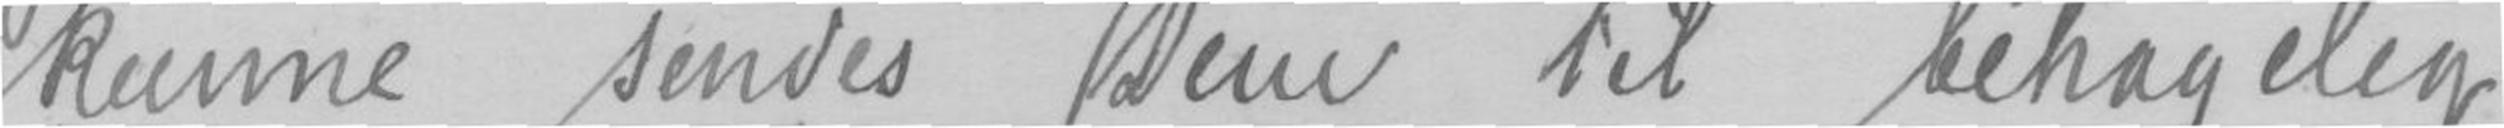kunne sendes Dem til behagelig
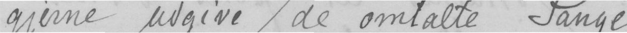gjerne udgive de omtalte Sange
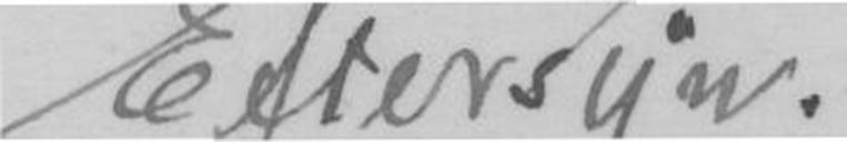Eftersyn.
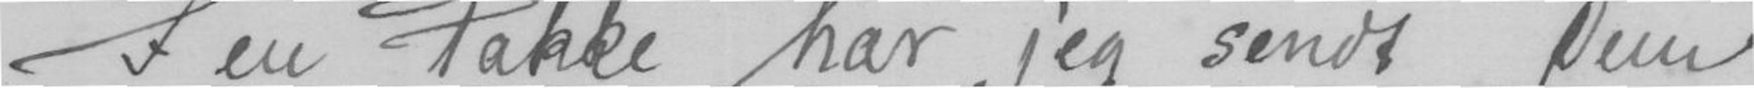I en pakke har jeg sendt Dem
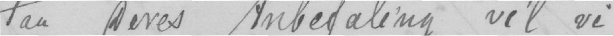Paa Deres Anbefaling vil vi
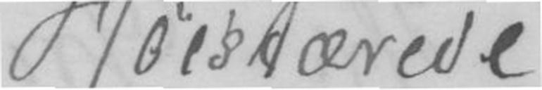Høistærede
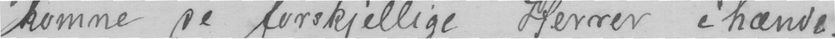komme De forskjellige Herrer i hænde.
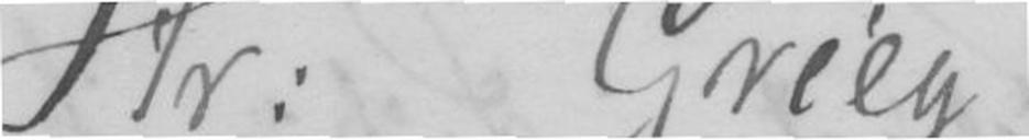Hr. Grieg!
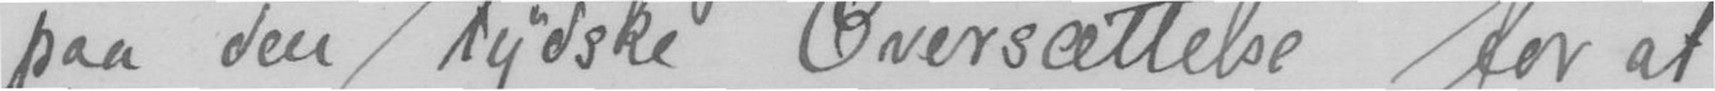paa den tydske Oversættelse for at
